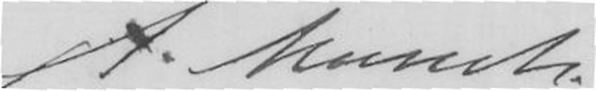A. Munch.
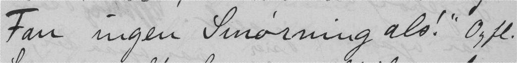Fan ingen Smørning als!" Og fl.
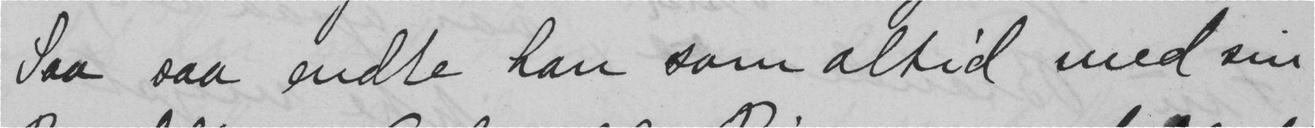Saa saa endte han som altid med sin
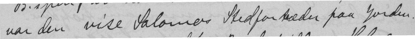var den vise Salomos Stedfortæder paa Jorden.
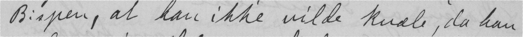Bispen, at han ikke vilde knæle, da han
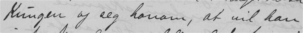Kungen og sej honom, at vil han
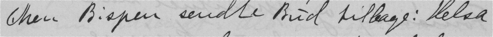Men Bispen sendte Bud tilbage: Helsa
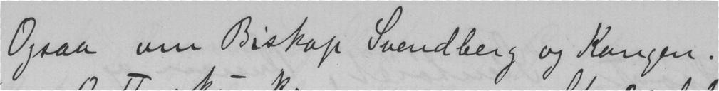Ogsaa om Biskop Svendberg og Kongen.
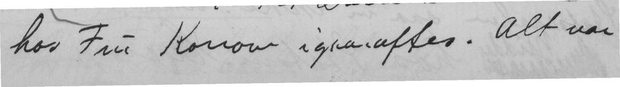hos Fru Konow igaaraftes. Alt var
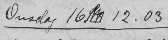Onsdag 16. 12.03
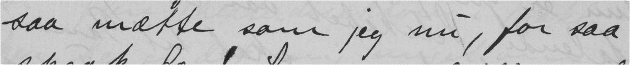saa mætte som jeg nu, for saa
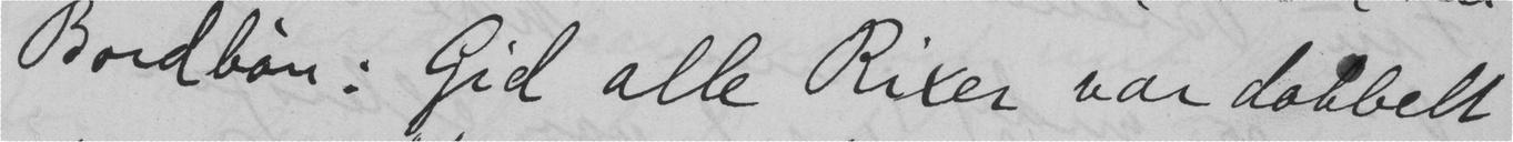Bordbøn: Gid alle Rixer var dobbelt
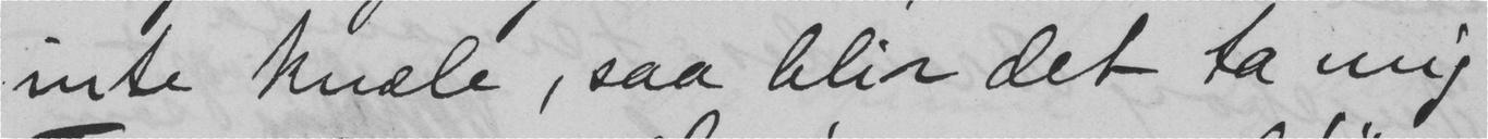inte knæle, saa blir det ta mig
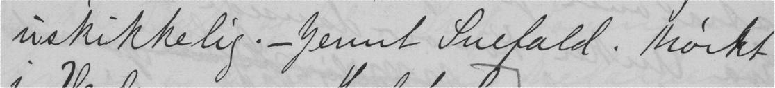uskikkelig. -Jevnt Snefald. Mørkt
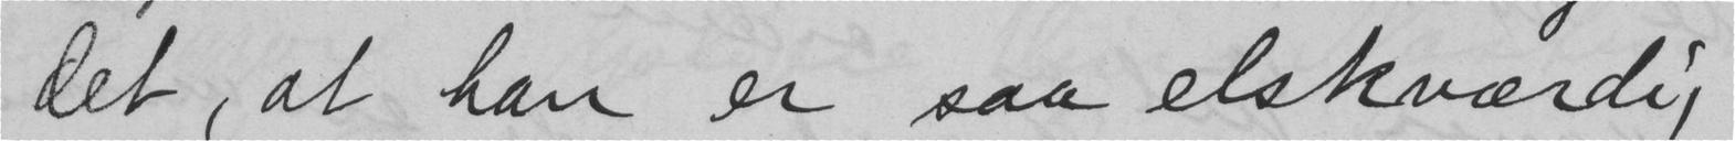det, at han er saa elskværdig
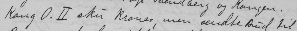Kong O. II sku Krones; men sendte Bud til
